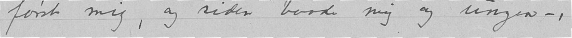først mig, og siden baade mig og ungen -,
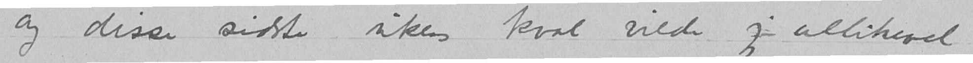og disse sidste ukers kval vilde jeg allikevel
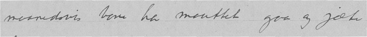maanedsvis bare har maattet gaa og jæte
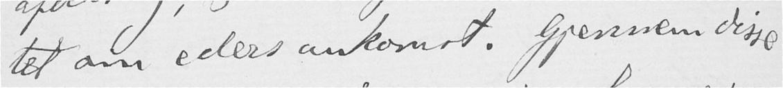tet om eders ankomst. Gjennem disse
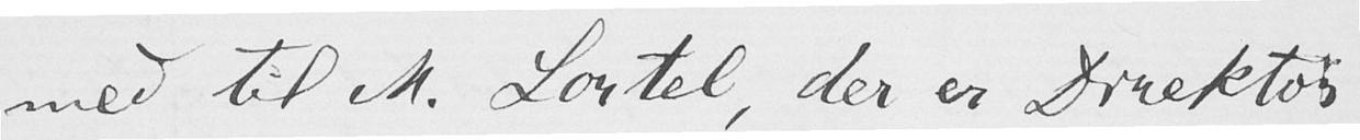med til M. Lortel, der er Direktør
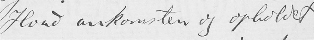Hvad ankomsten og opholdet
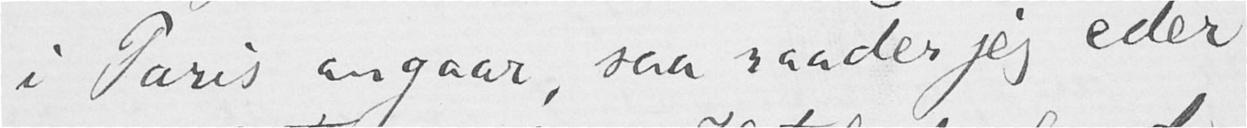i Paris angaar, saa raader jeg eder
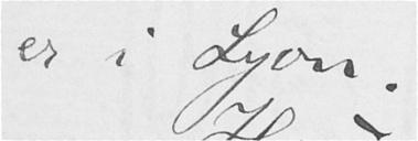er i Lyon.
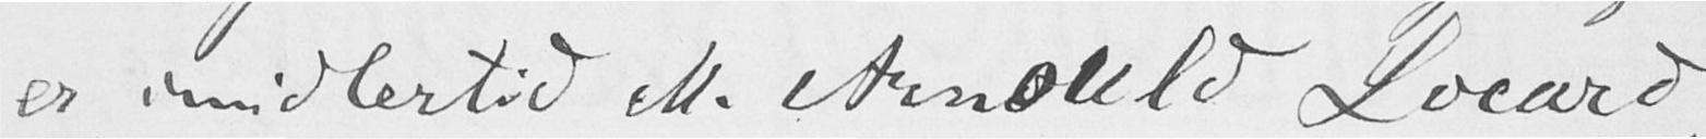er imidlertid M. Arnould Locard,
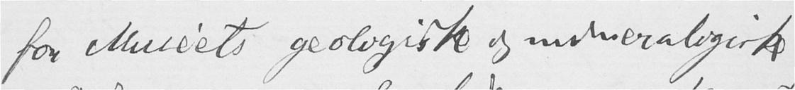for Muséets geologiske og mineralogiske
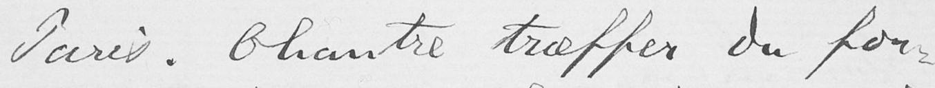Paris. Chantre træffer du for-
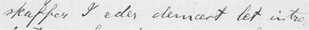skaffer I eder dernæst let intro-
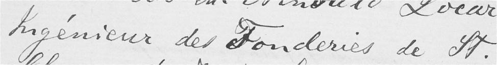Ingénieur des Fonderies de St.
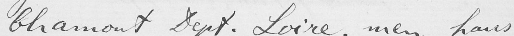Chamont, Dept. Loire; men hans
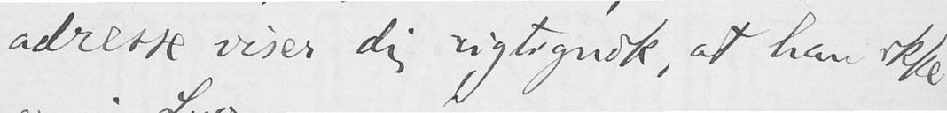adresse viser dig rigtignok, at han ikke
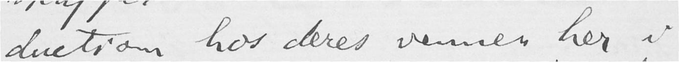duction hos deres venner her i
